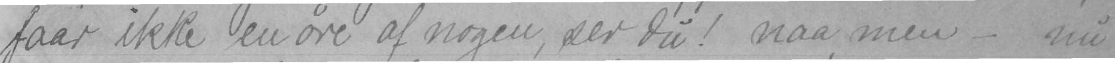faar ikke en øre af nogen, ser du! naa, men - nu
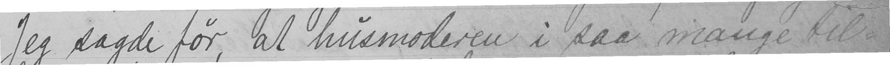Jeg sagde før, at husmoderen i saa mange til-
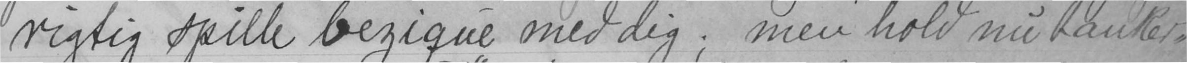rigtig spille begique med dig; men hold nu tanker-
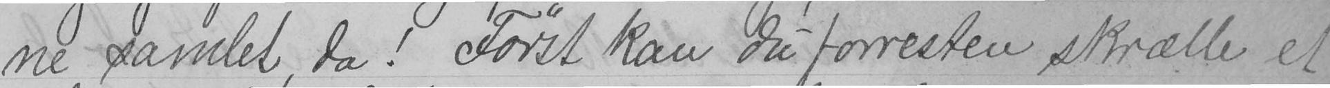ne samlet, da! Først kan du forresten skrælle et
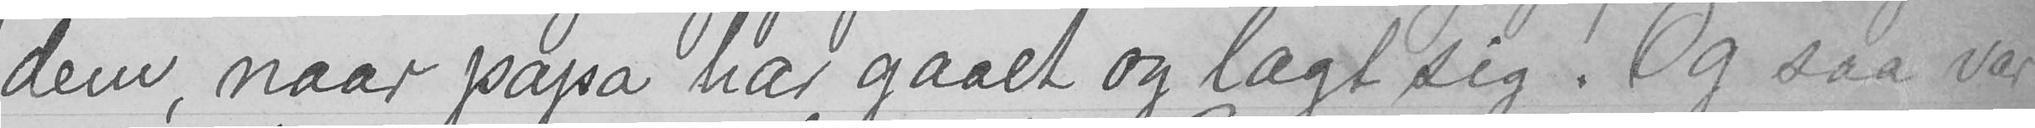dem, naar papa har gaaet og lagt sig. Og saa var
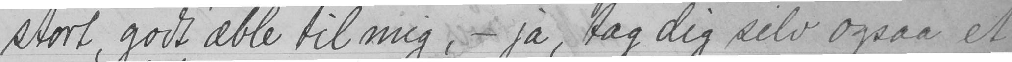stort, godt æble til mig, - ja, tag dig selv ogsaa et
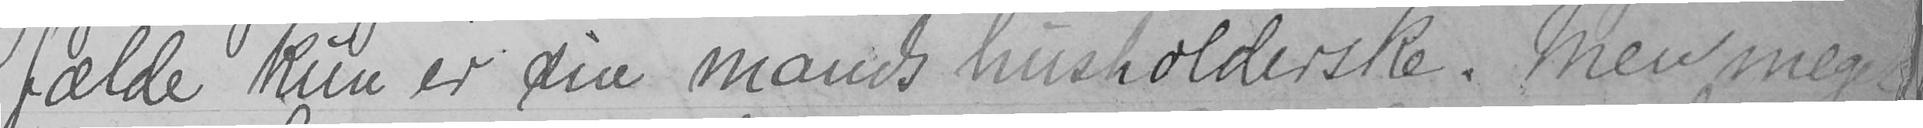fælde kun er sin mands husholderske. Men meget
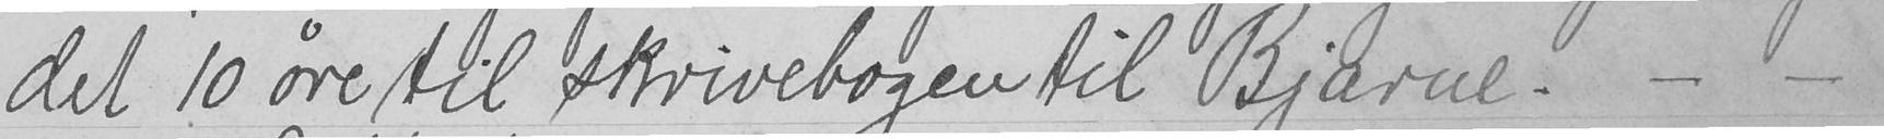det 10 øre til skrivebogen til Bjarne. - -
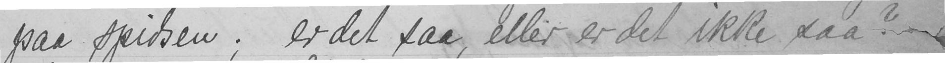paa spidsen; er det saa, eller er det ikke saa?
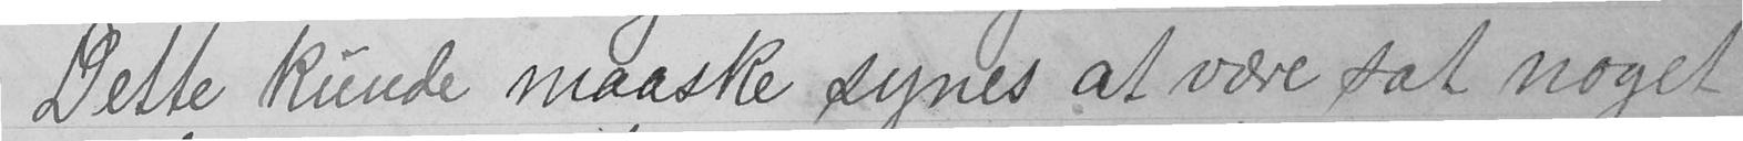Dette kunde maaske synes at være sat noget
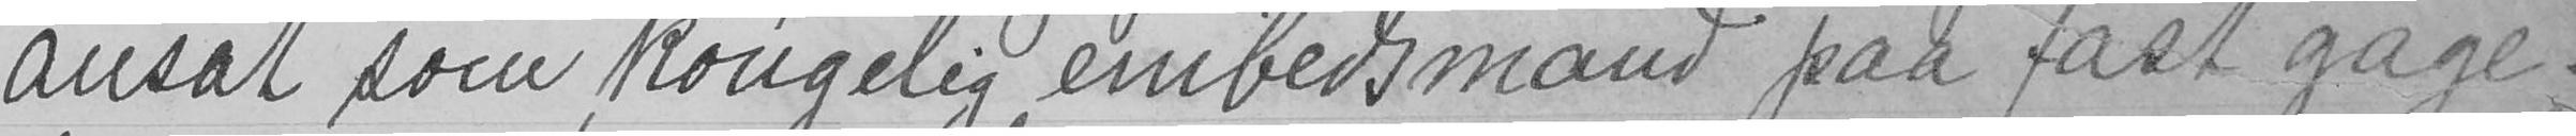ansat som kongelig embedsmand paa fast gage.
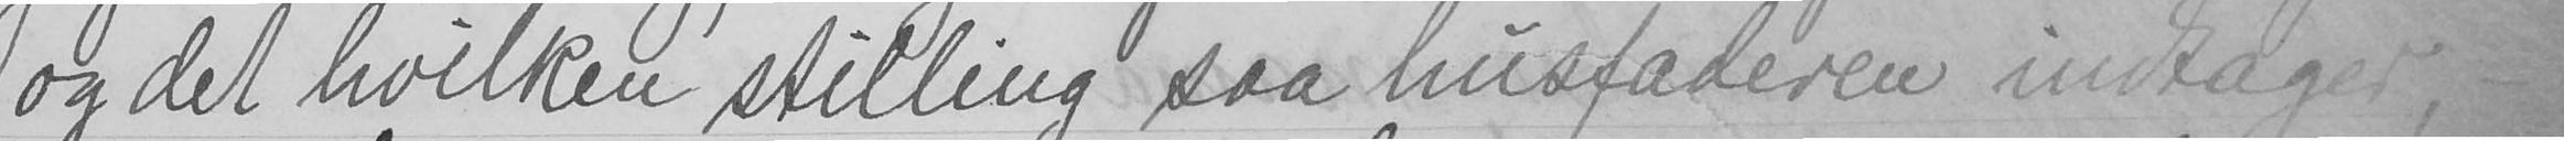og det hvilken stilling saa husfaderen indtager,
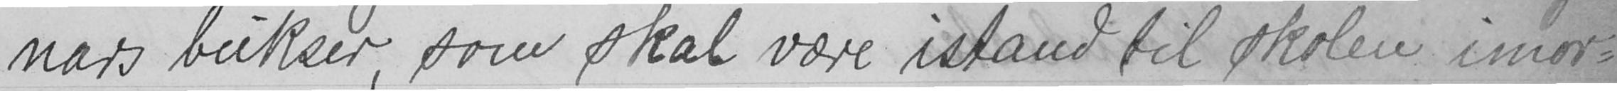nars bukser, som skal være istand til skolen imor-
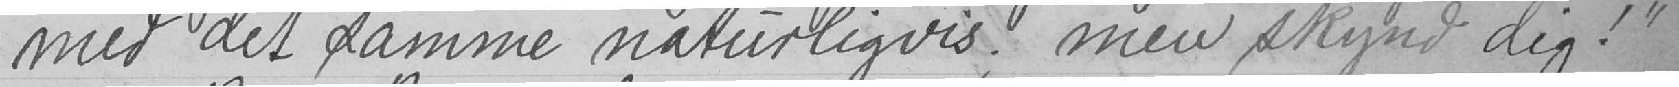med det samme naturligvis; men skynd dig!"
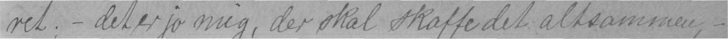ret; - det er jo mig, der skal skaffe det altsammen, -
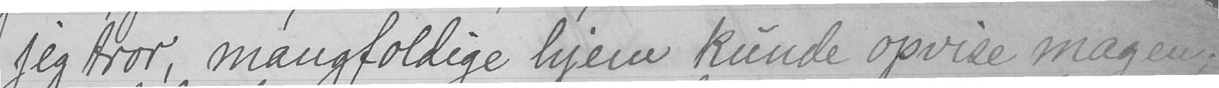jeg tror, mangfoldige hjem kunde opvise magen;
